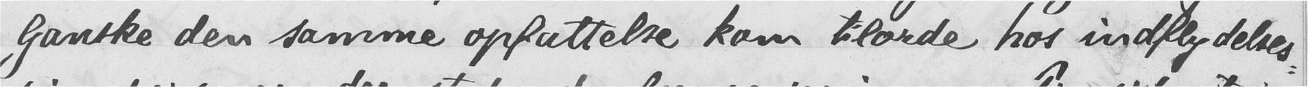Ganske den samme opfattelse kom tilorde hos indflydelses-
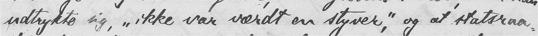udtrykte sig, "ikke var værdt en styver", og at statsraa-
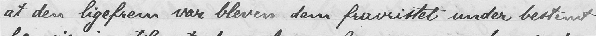at den ligefrem var bleven dem fravristet under bestemt
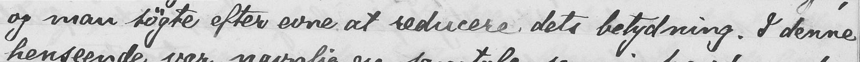og man søgte efter evne at reducere dets betydning. I denne
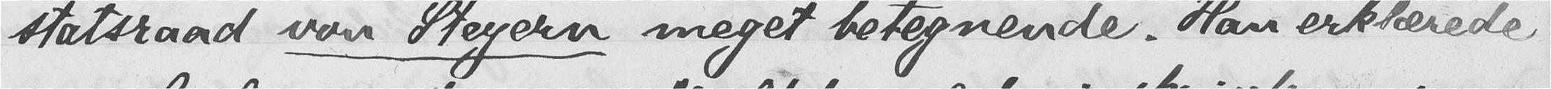statsraad von Steyern meget betegnende. Han erklærede
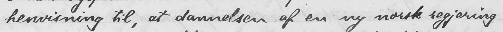henvisning til, at dannelsen af en ny norsk regjering
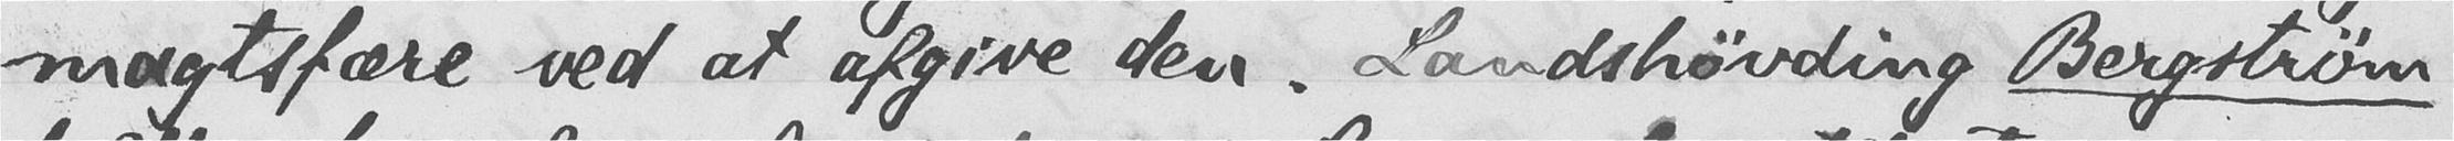magtsfære ved at afgive den. Landshøvding Bergström
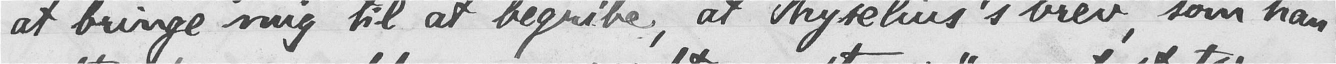at bringe mig til at begribe, at Thyselius's brev, som han
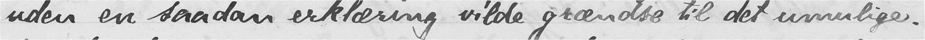uden en saadan erklæring vilde grændse til det umulige.
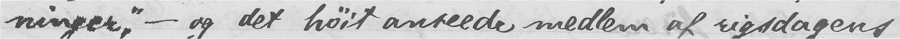ninger," - og det høit anseede medlem af rigsdagens
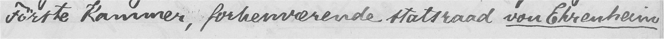Første Kammer, forhenværende statsraad von Ehrenheim
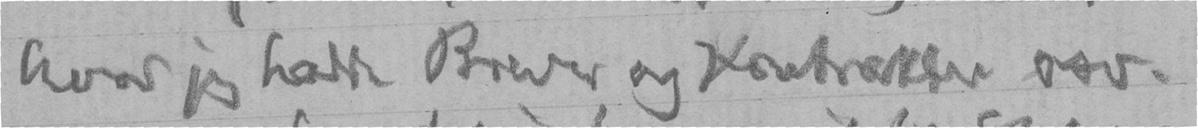hvor jeg hadde Brever og Kontrakter osv.
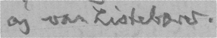og var Listebærer.
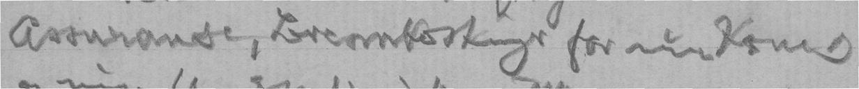Assuranse, Leveomkostninger for min Kone og
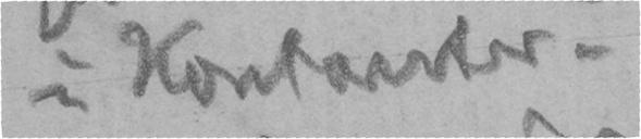i Kontanter.
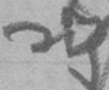sig
